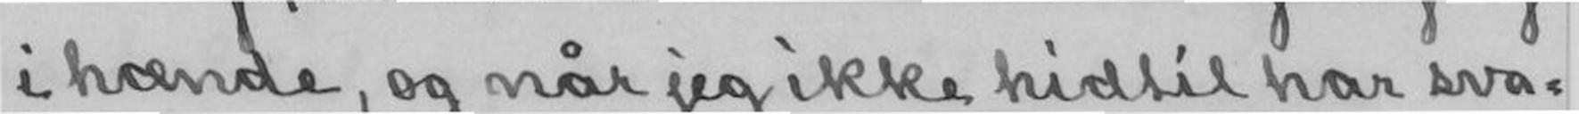i hænde, og når jeg ikke hidtil har sva-
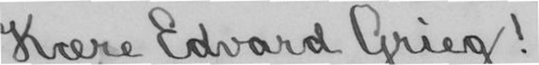Kære Edvard Grieg!
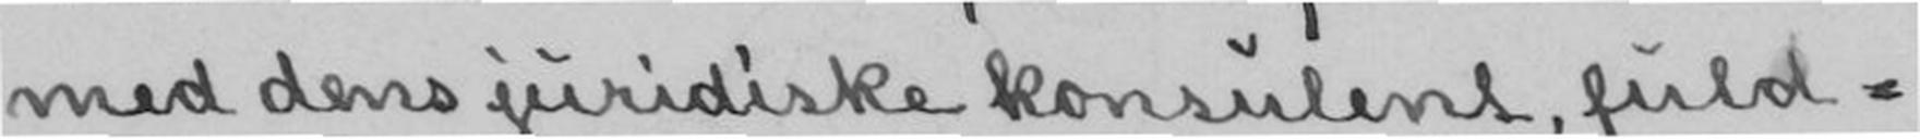med dens juridiske konsulent, fuld-
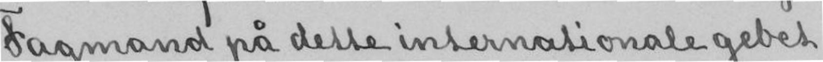Fagmand på dette internationale gebet
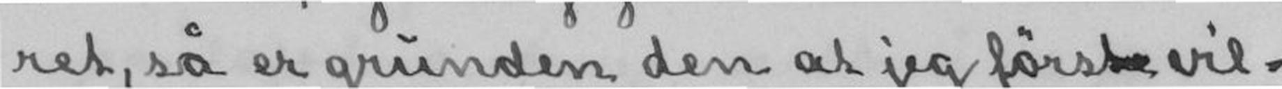ret, så er grunden den at jeg først vil-
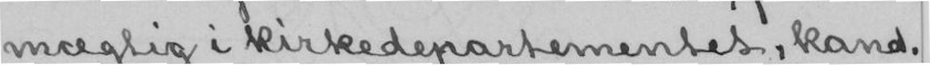mægtig i kirkedepartementet, kand.
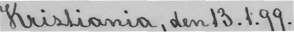Kristiania, den 13.1.99.
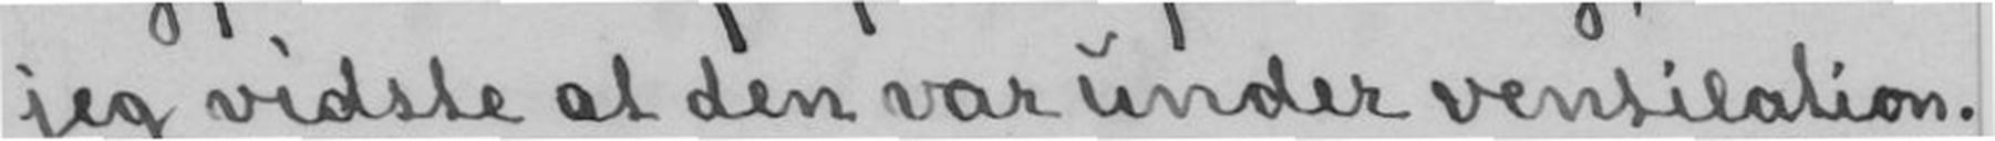jeg vidste at den var under ventilation.
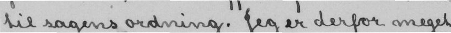til sagens ordning. Jeg er derfor meget
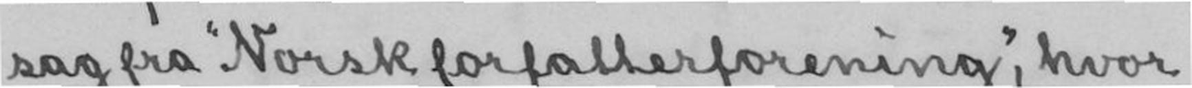sag fra "Norsk forfatterforening", hvor
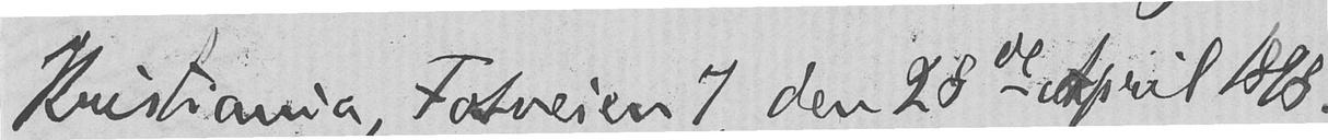Kristiania, Fossveien 7, den 28de April 1898.
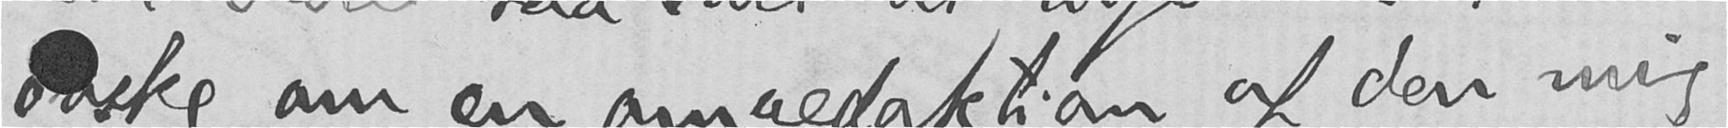ønske om en omredaktion af den mig
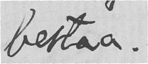bestaa.
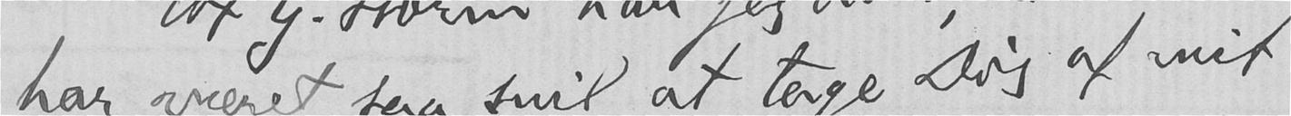har været saa snil at tage Dig af mit
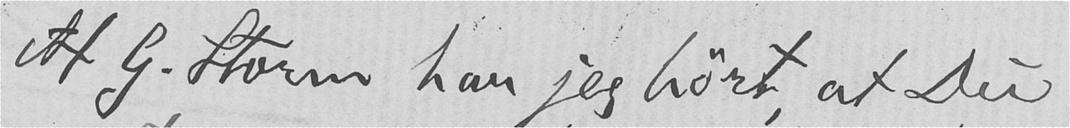Af G. Storm har jeg hørt, at Du
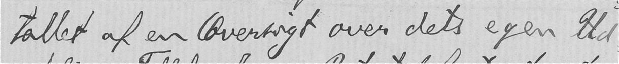tallet af en Oversigt over dets egen Ud-
